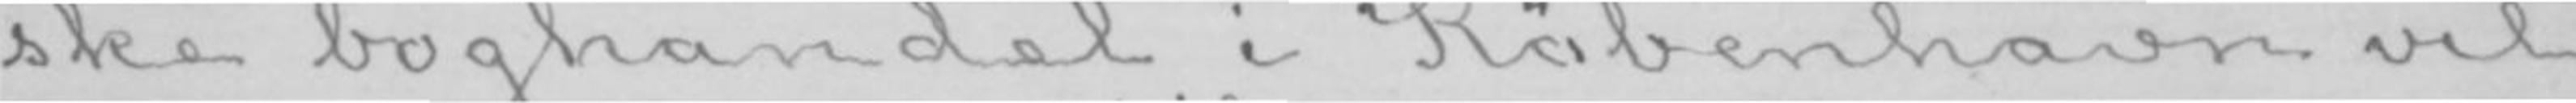ske boghandel i København vil
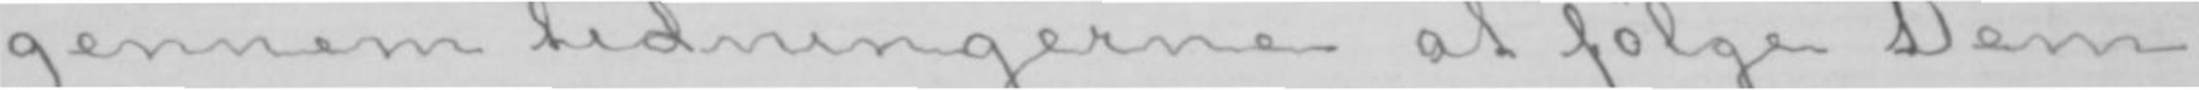gennem tidningerne at følge Dem
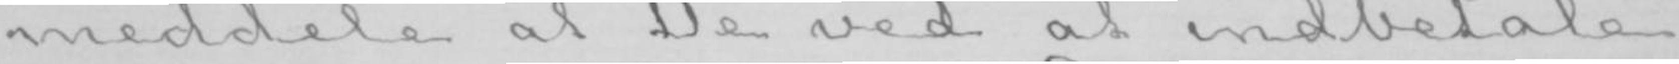meddele at De ved at indbetale
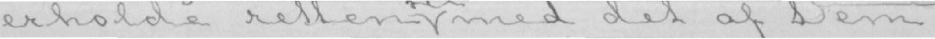erholde retten til med det af Dem
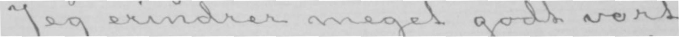Jeg erindrer meget godt vort
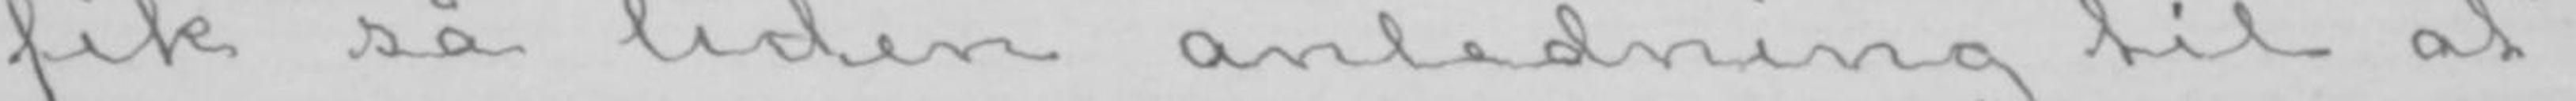fik så liden anledning til at
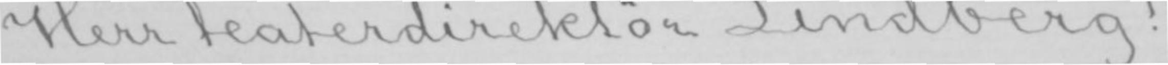Herr teaterdirektør Lindberg!
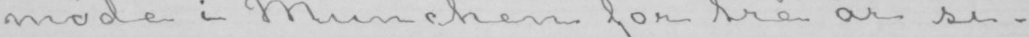møde i München for tre år si-
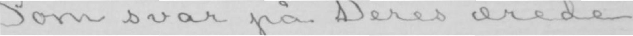Som svar på Deres ærede
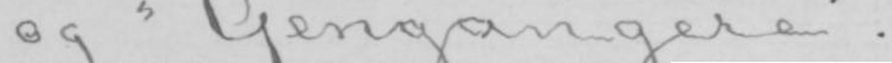og «Gengangere».
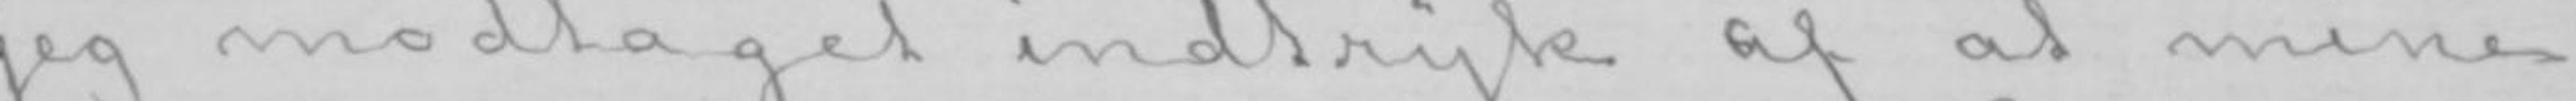jeg modtaget indtryk af at mine
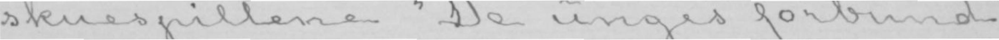skuespillene «De unges forbund»
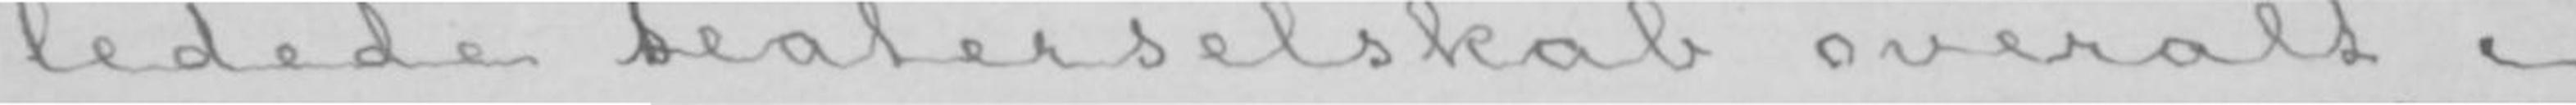ledede teaterselskab overalt i
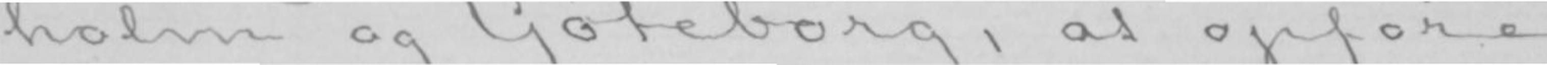holm og Göteborg, at opføre
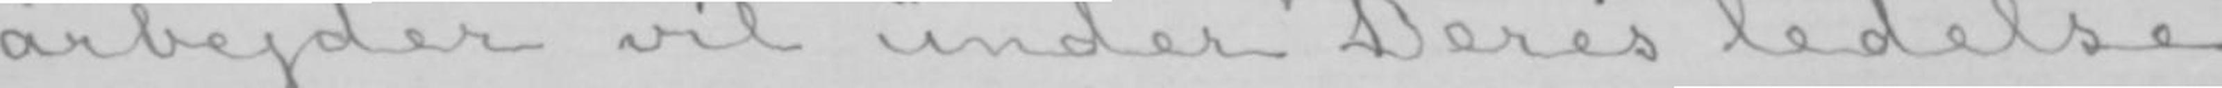arbejder vil under Deres ledelse
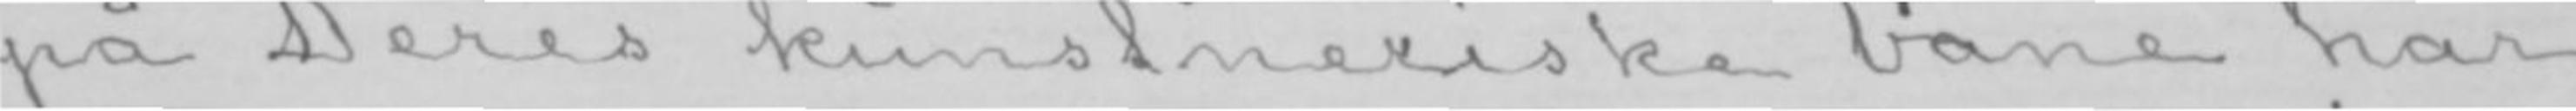på Deres kunstneriske bane har
